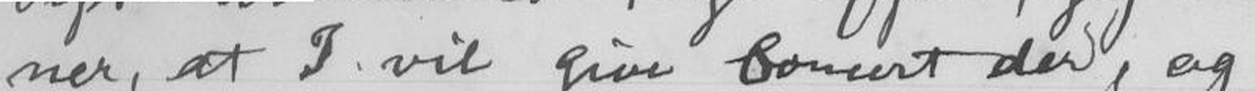ner, at I vil give concert der, og
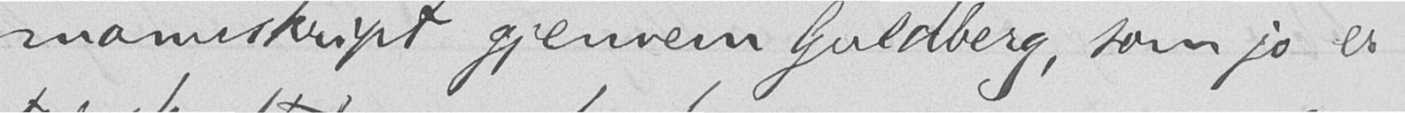manuskript gjennem Guldberg, som jo er
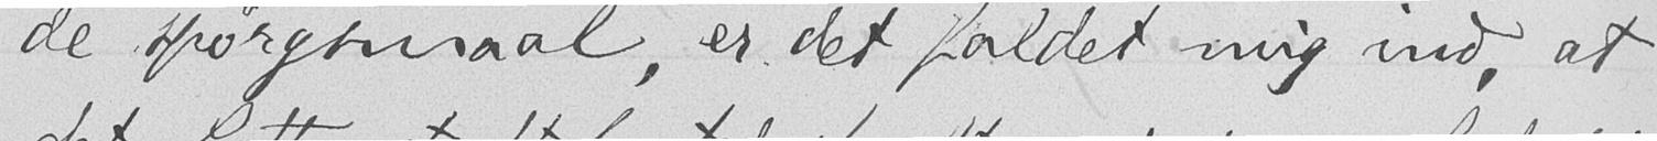de spørgsmaal, er det faldet mig ind, at
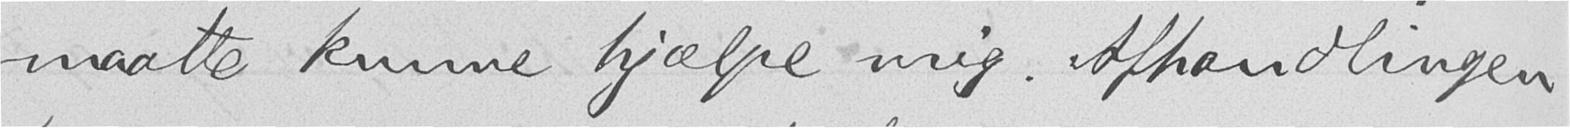maatte kunne hjælpe mig. Afhandlingen
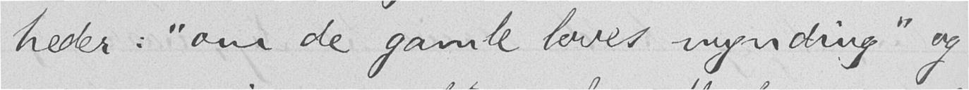heder: "om de gamle loves mynding" og
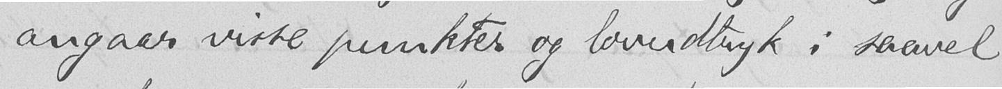angaar visse punkter og lovudtryk i saavel
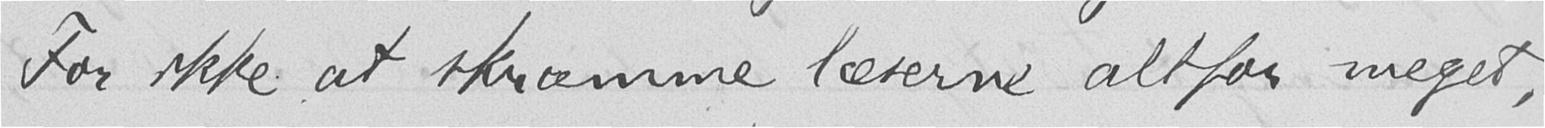For ikke at skræmme læserne altfor meget,
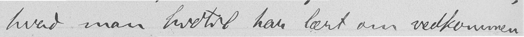hvad man hidtil har lært om vedkommen-
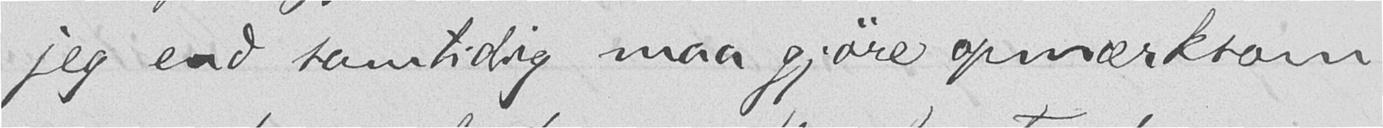jeg end samtidig maa gjøre opmærksom
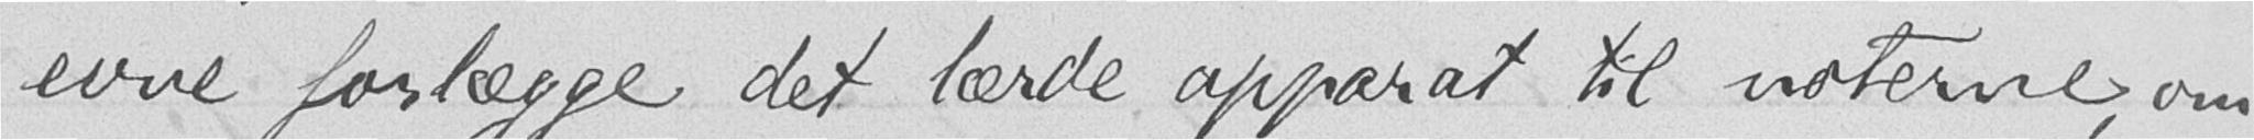evne forlægge det lærde apparat til noterne, om
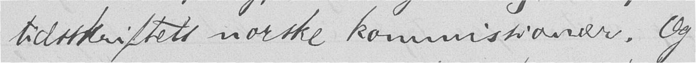tidsskriftets norske kommissionær. Og
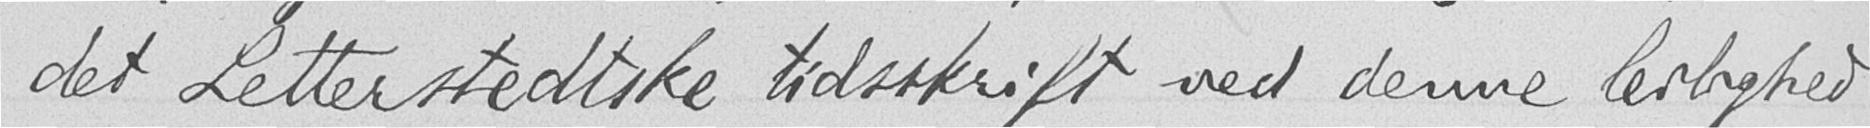det Letterstedtske tidsskrift ved denne leilighed
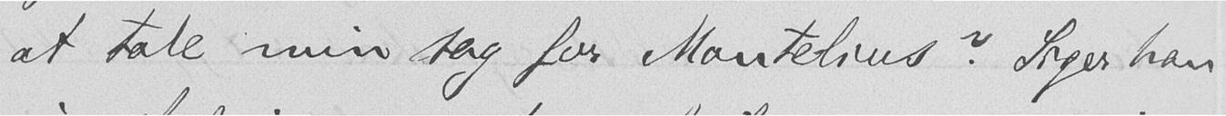at tale min sag for Montelius? Siger han
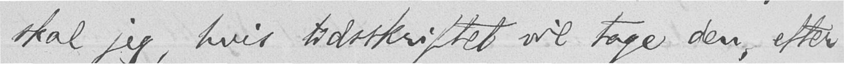skal jeg, hvis tidsskriftet vil tage den, efter
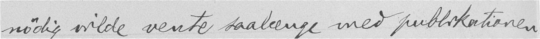nødig vilde vente saalænge med publikationen
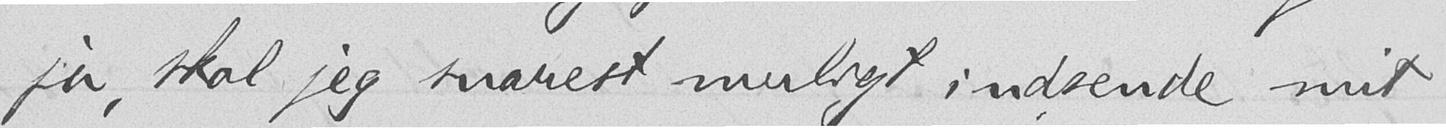ja, skal jeg snarest muligt indsende mit
